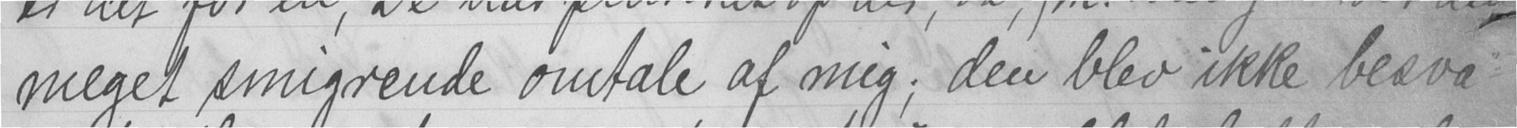meget smigrende omtale af mig; den blev ikke besva-
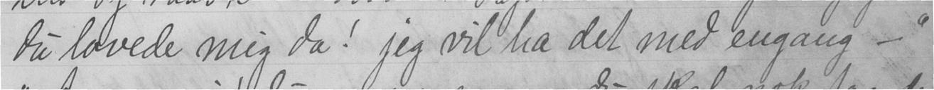du lovede mig da! jeg vil ha det med engang - "
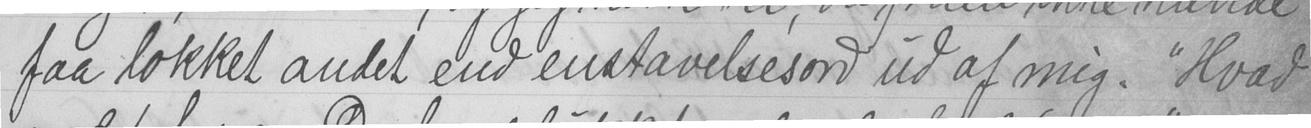faa lokket andet end enstavelsesord ud af mig. "Hvad
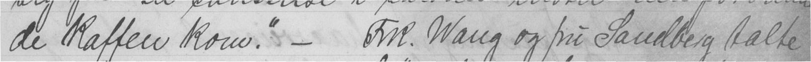de kaffen kom." - Frk. Wang og fru Sandberg talte
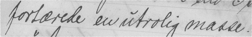fortærede en utrolig masse.
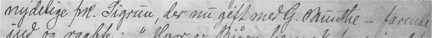nydelige frk. Sigrun, der nu gift med G. Munthe - farende
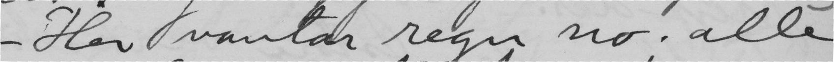Her vantar regn no; alle
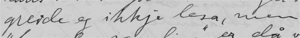greide eg ikkje lesa, men
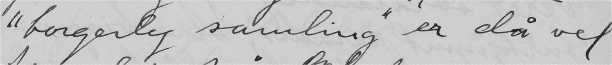"borgerleg samling" er då vel
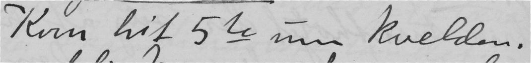Kom hit 5te um kvelden.
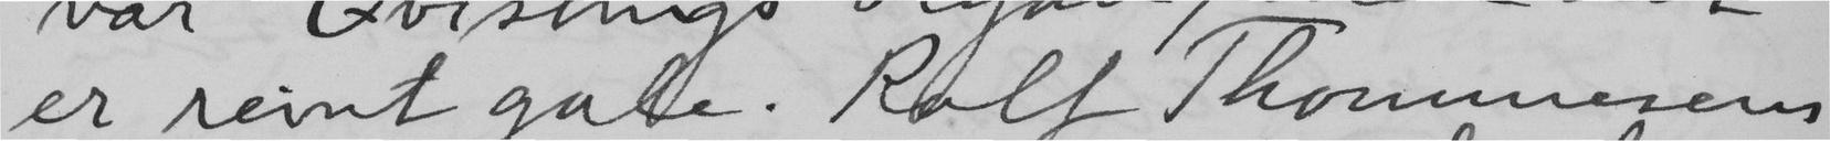er reint gale. Rolf Thommesens
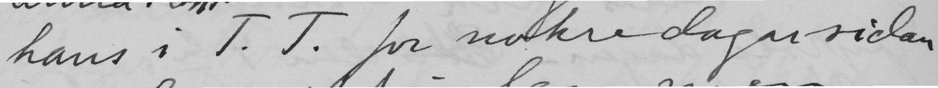hans i T.T. for nokre dagar sidan
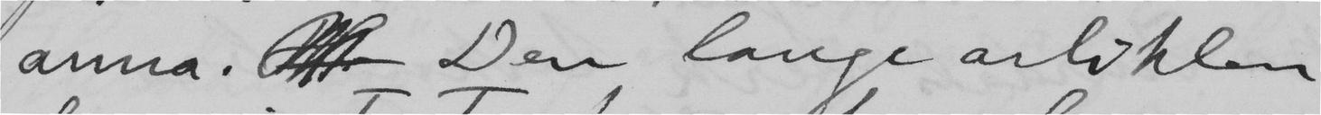anna.  Den lange artiklen
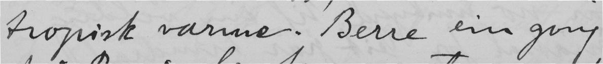tropisk varme. Berre ein gong
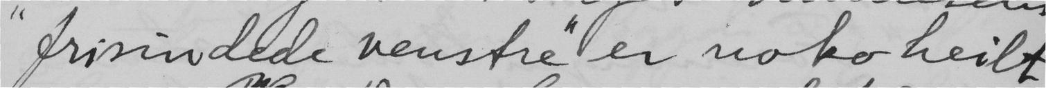"frisindede venstre" er noko heilt
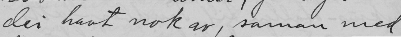dei havt nok av, saman med
